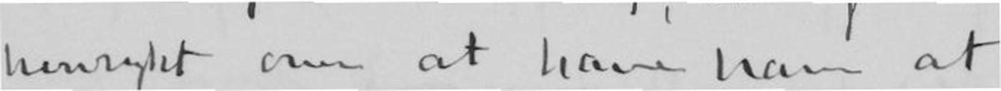henrykt over at have ham at
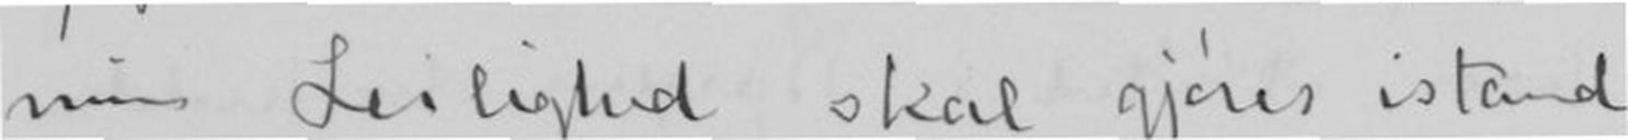min Leilighed skal gjøres istand,
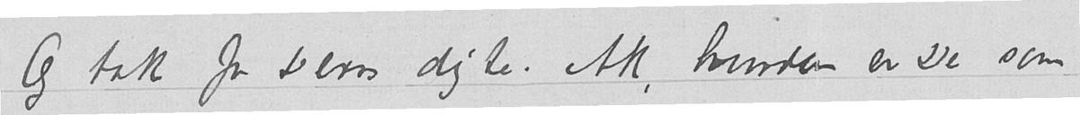Og tak for Deres digte. Ak, hvordan er De som
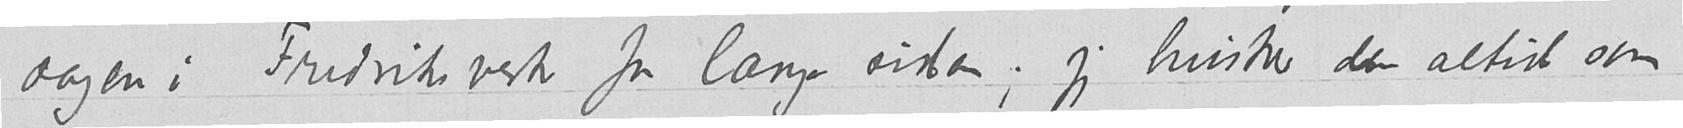dagen i Fredviksverk for længe siden; jeg husker den altid som
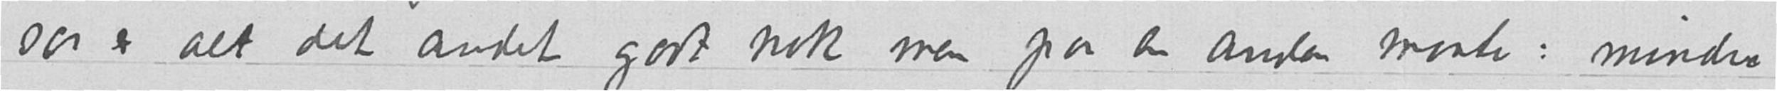saa er alt det andet godt nok men paa en anden maate: mindre
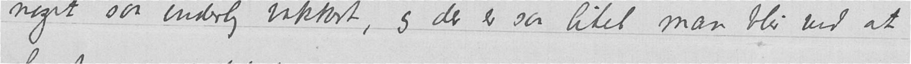noget saa inderlig vakkert, og der er saa litet man blir ved at
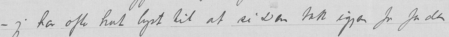- jeg har ofte hat lyst til at si Dem tak igjen for for den
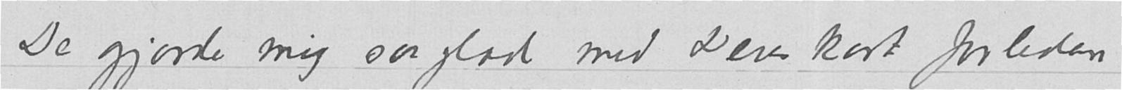De gjorde mig saa glad med Deres kort forleden
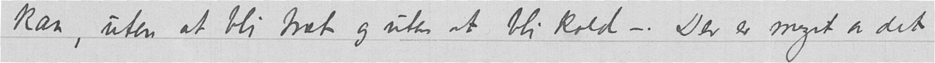kan, uten at bli træt og uten at bli kold –. Der er meget av det
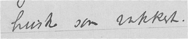huske som vakkert.
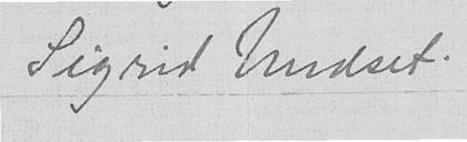Sigrid Undset.
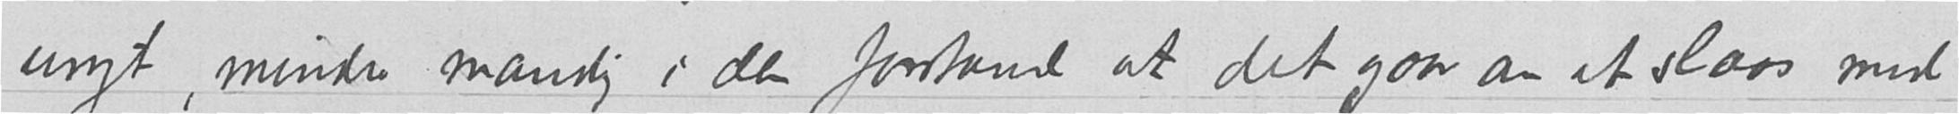ungt, mindre mandig i den forstand at det gaar an at slaas med
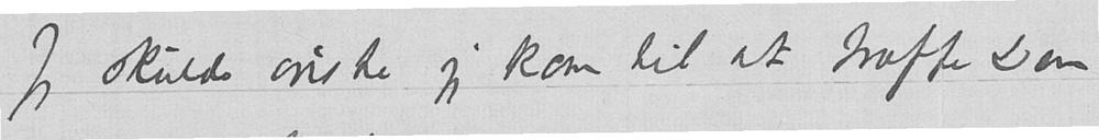Jeg skulde ønske jeg kom til at træffe Dem
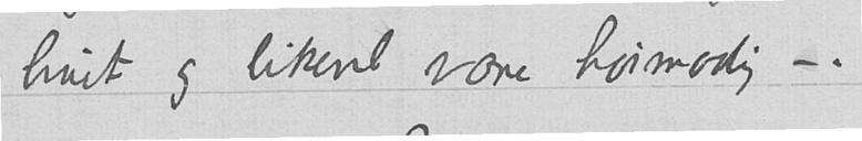livet og likevel være høimodig -.
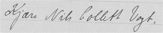Kjære Nils Collett Vogt,
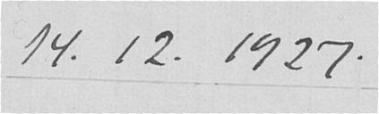14.12.1927.
